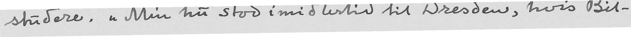studere. "Min hu stod imidlertid til Dresden, hvis Bil-
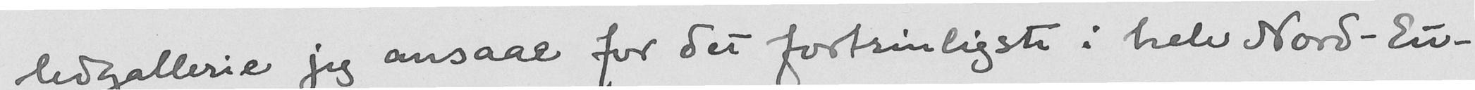ledgallerie jeg ansaae for det fortrinligste i hele Nord-Eu-
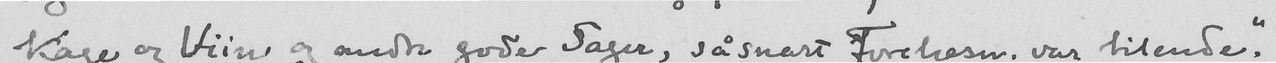kage og Viin og andre gode Sager, såsnart Forelæsn. var tilende."
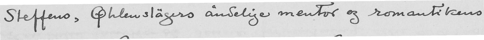Steffens, Øhlenslägers åndelige mentor og romantikens
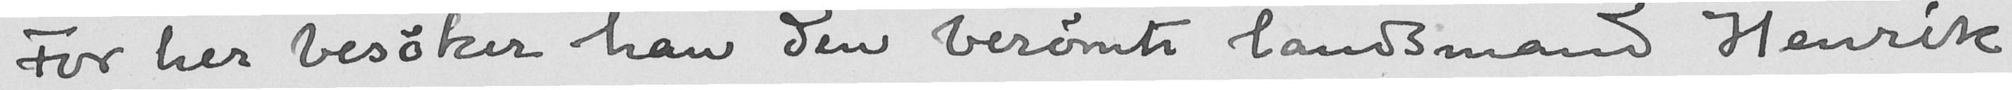For her besøker han den berømte landsmand Henrik
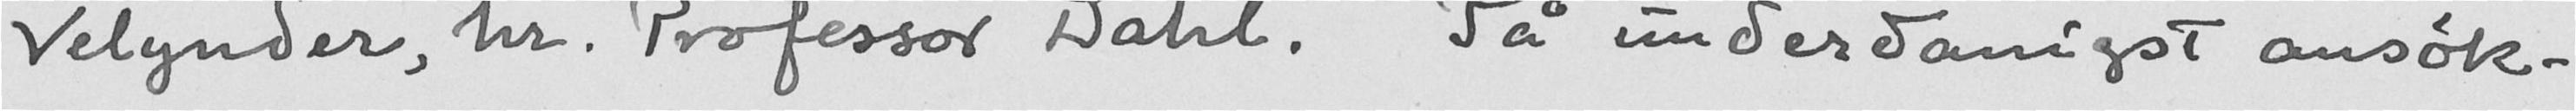Velynder, hr. Professor Dahl." Så underdanigst ansøk-
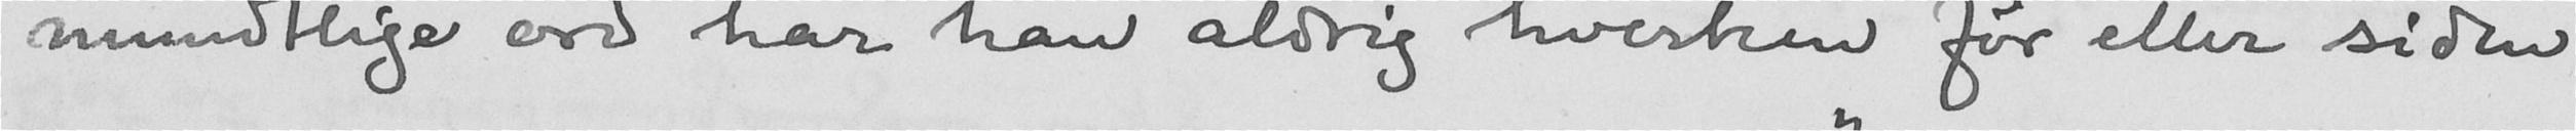mundtlige ord har han aldrig hverken før eller siden
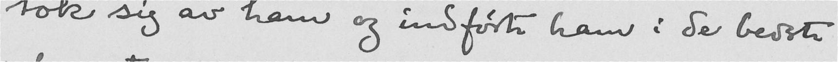tok sig av ham og indførte ham i de bedste
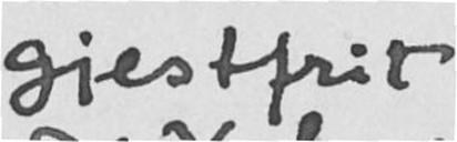gjestfrit
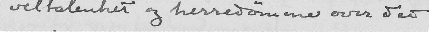veltalenhet og herredømme over det
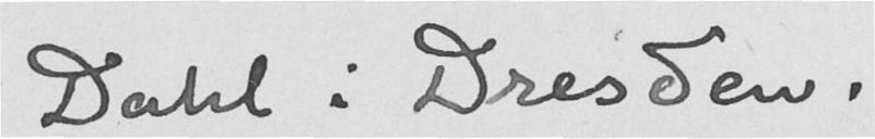Dahl i Dresden.
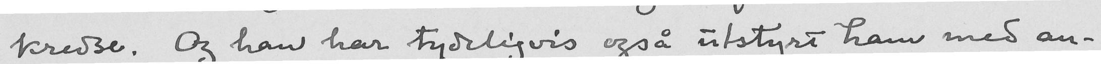kredse. Og han har tydeligvis også utstyrt ham med an-
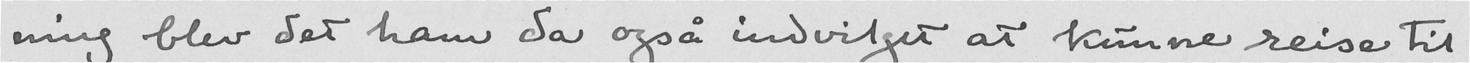ning blev det ham da også indvilget at kunne reise til
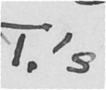T.'s
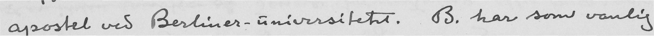apostel ved Berliner- universitetet. B. har som vanlig
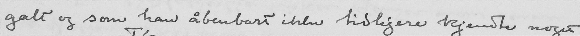galt og som han åbenbart ikke tidligere kjendte noget
